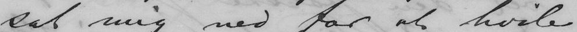sat mig ned for at hvile
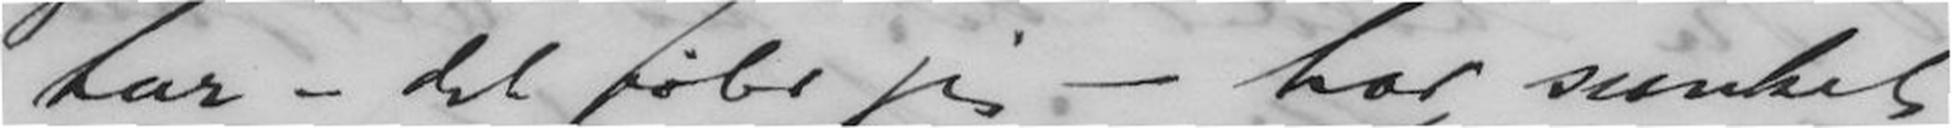tur - det føler jeg - har sunket
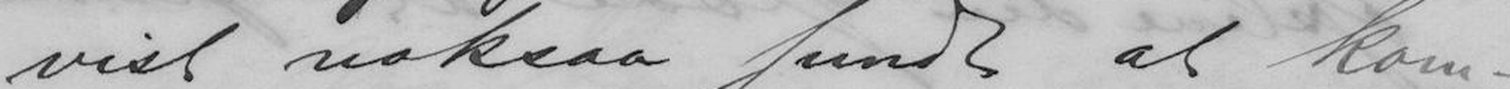vist noksaa sundt at kom-
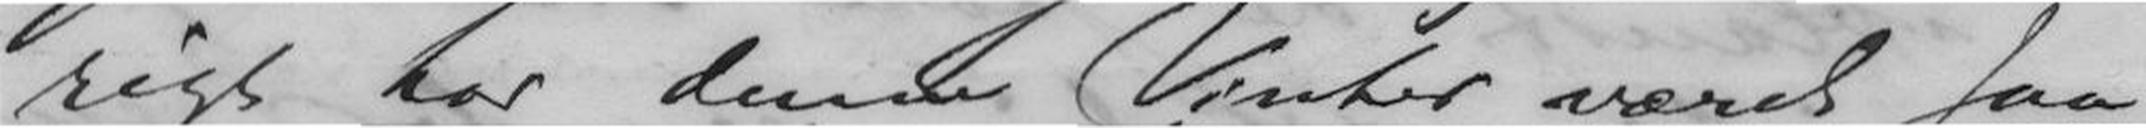rigt har denne Vinter været saa
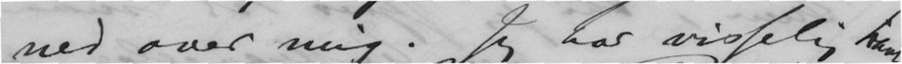ned over mig. Jeg har visselig havt
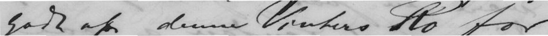godt af denne Vinters Ro for
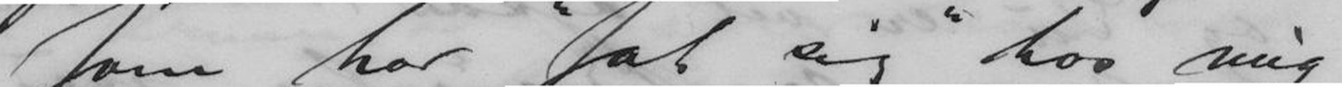som har sat sig hos mig -
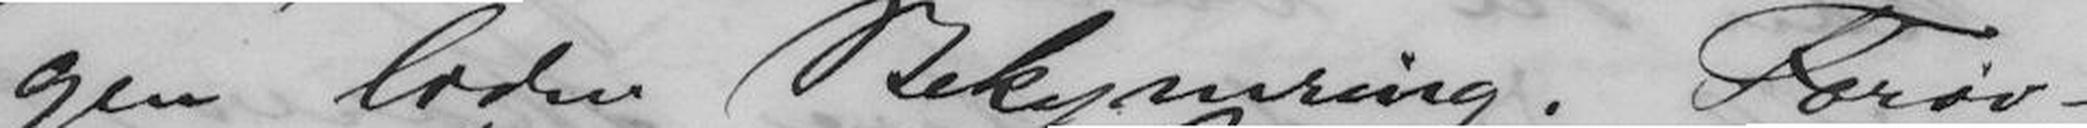gen liden Bekymring. Forøv-
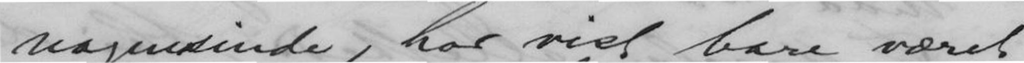nogensinde, har vist bare været
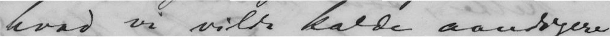hvad vi vilde kalde aandigere
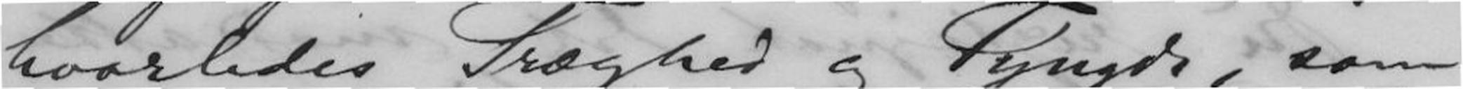hvorledes Træghed og Tyngde, som
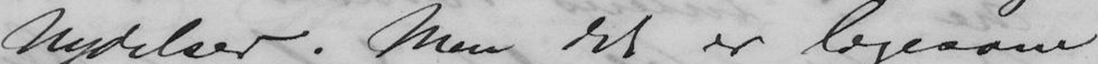Nydelser. Men det er ligesom
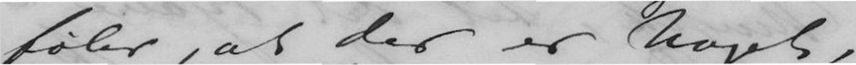føler, at der er Noget,
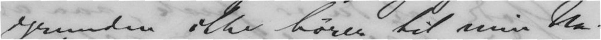igrunden ikke hører til min Na-
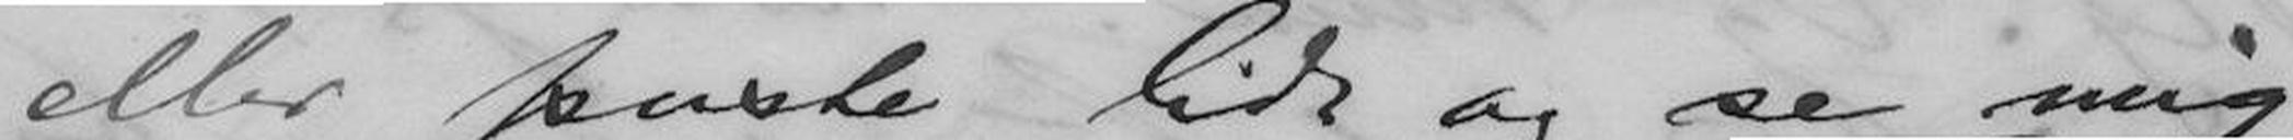eller puste lidt og se mig
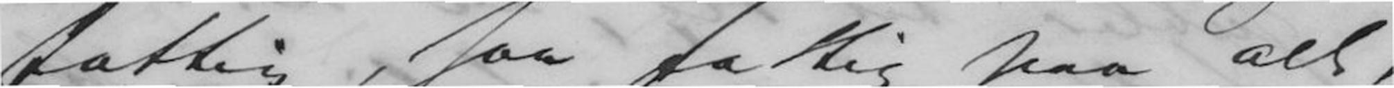fattig, saa fattig paa alt,
